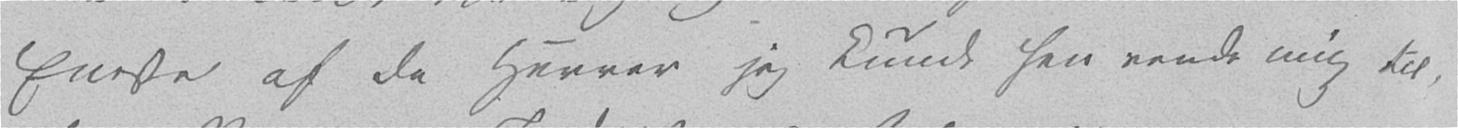Eneste af de Herrer jeg kunde hen vende mig til,
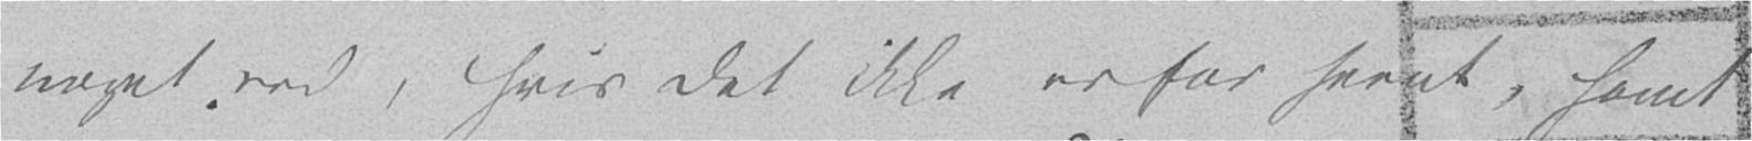noget ved, hvis det ikke er for seent, samt
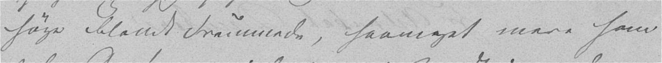søge blandt Fremmede, saameget mere som
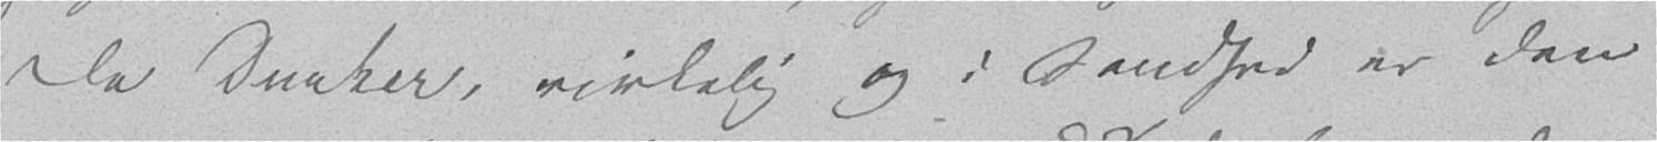De Dunker, virkelig og i Sandhed er den
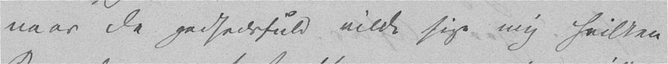naar De godhedsfuld vilde sige mig hvilken
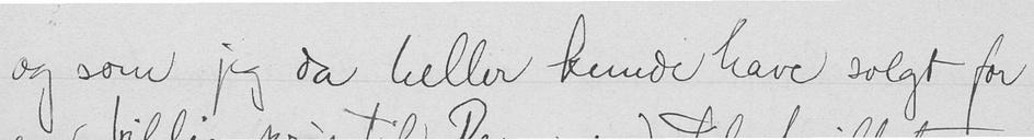og som jeg da heller kunde have solgt for
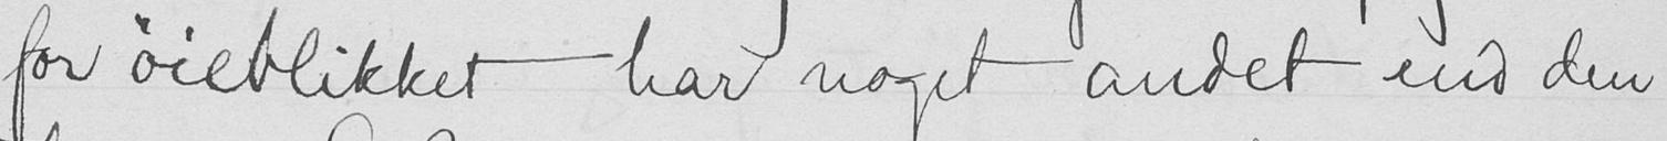for øieblikket har noget andet end den
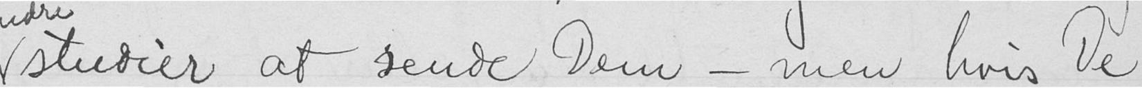studier at sende Dem - men hvis De
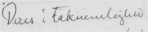Deres i taknemlighed
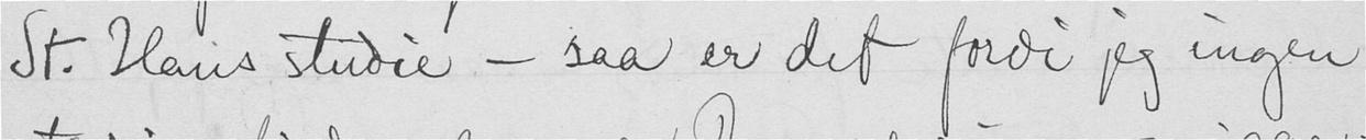St. Hans studie - saa er det fordi jeg ingen
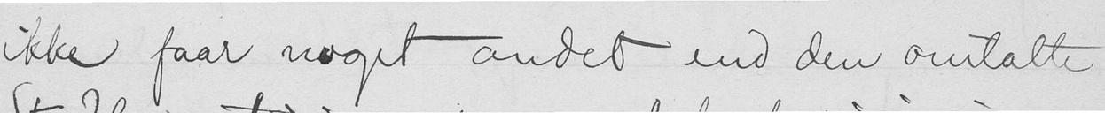ikke faar noget andet end den omtalte
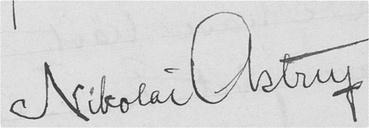Nikolai Astrup
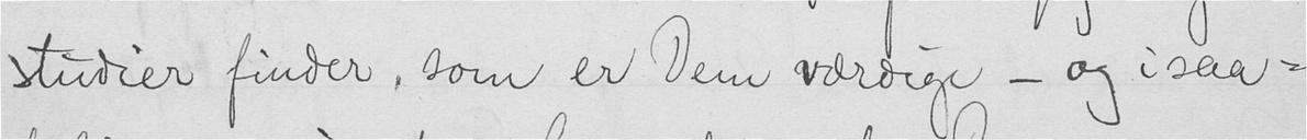studier finder, som er Dem værdige - og isaa-
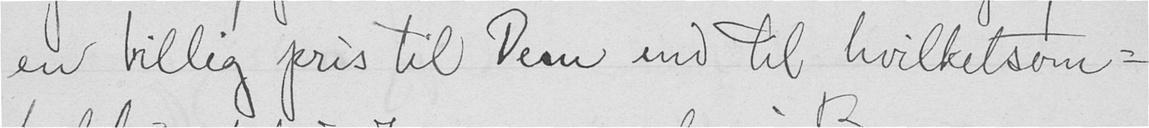en billig pris til Dem end til hvilketsom-
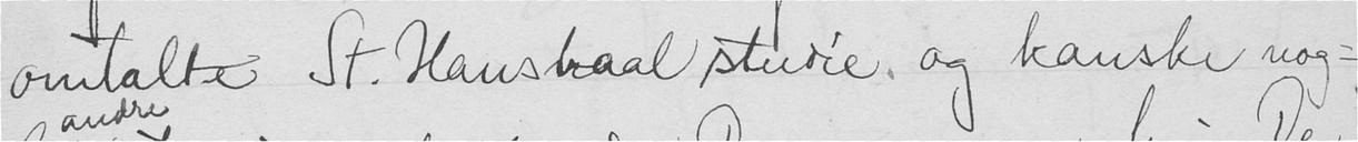omtalte St. Hansbaal studie og kanske nog-
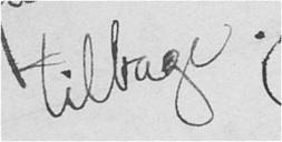tilbage.
